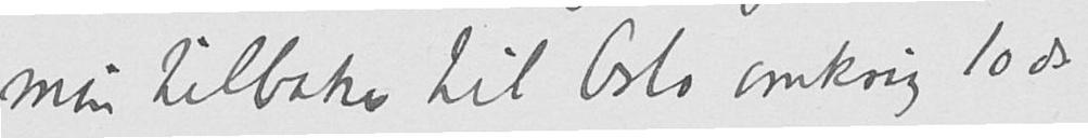min tilbake til Oslo omkrig 10 ds.
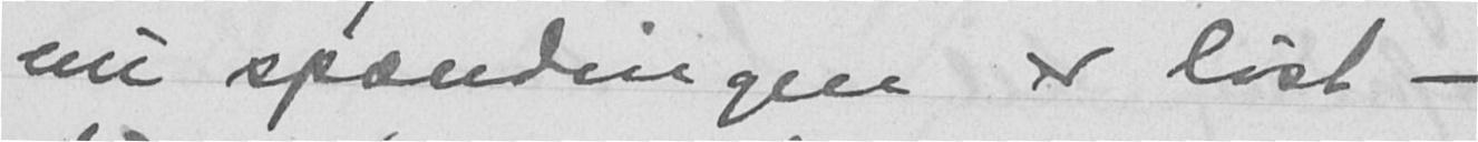nu spændingen er løst -
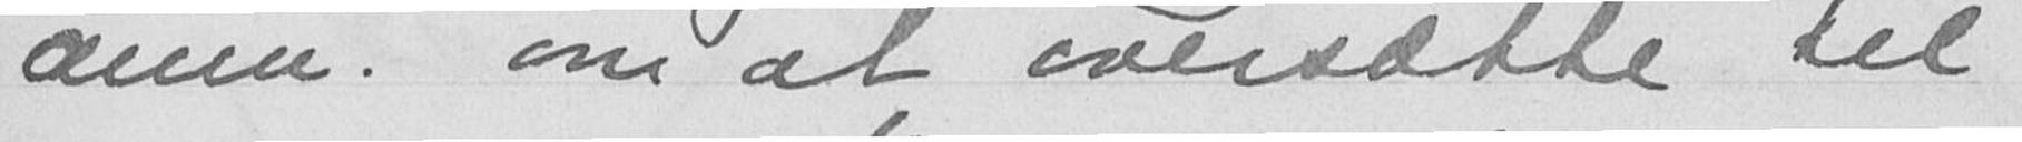anm. om at oversætte til
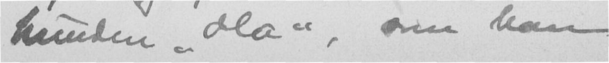hunden "Ola", som han
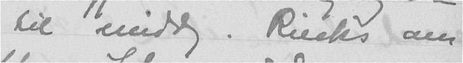til middag. Riecks om
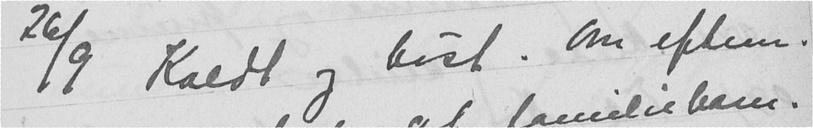26/9 Koldt og høst. Om efterm.
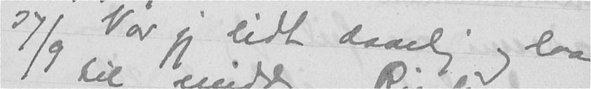27/9 Var jeg lidt daarlig og laa
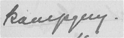kampgang.
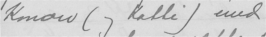Konow (og Katti) med
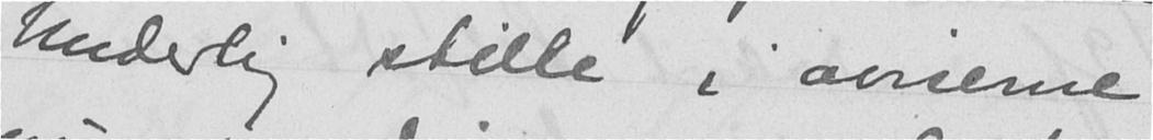Underlig stille i aviserne
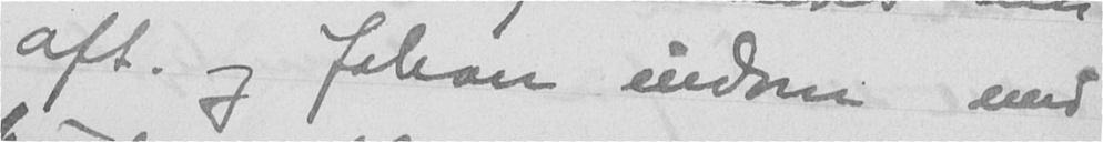aft. og Johan indom med
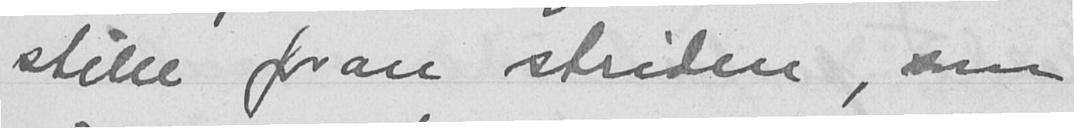stille foran striden, som
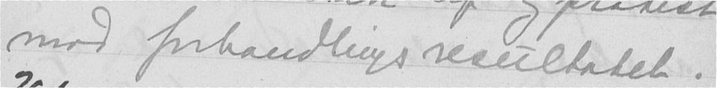mod forhandlingsresultatet.
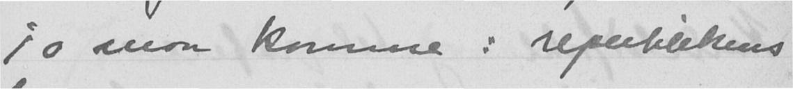jo maa komme: republikens
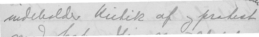indeholder kritik af og protest
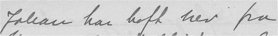Johan har haft brev fra
